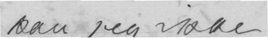kan jeg ikke
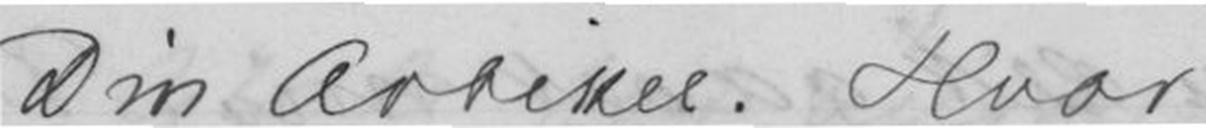Din Artikkel. Hvor
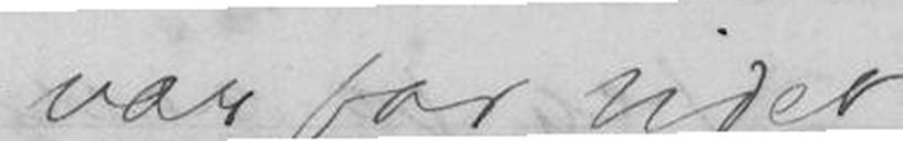var for lidet
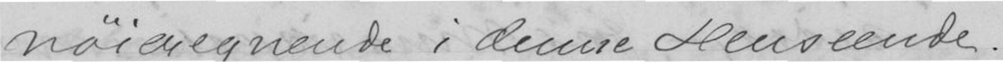nøieregnende i denne Henseende.
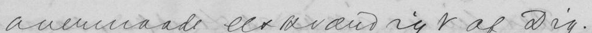overmaade elskværdigt af Dig.
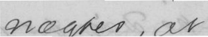nægtes, at
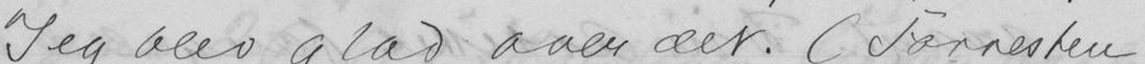Jeg blev glad over det. (Forresten
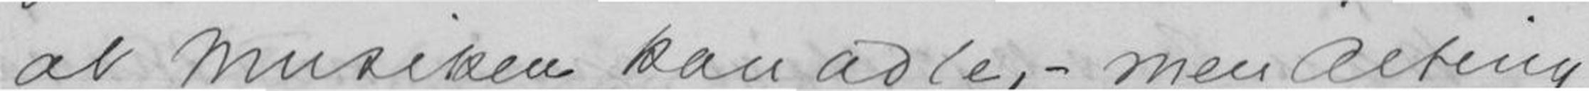at Musiken kan ad,- men Alting
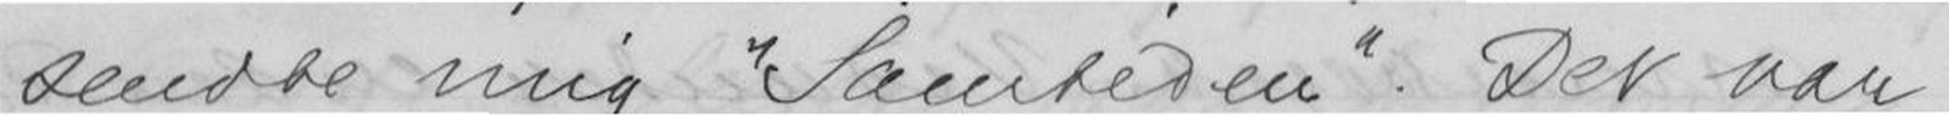sende mig "Sannheden" Det var
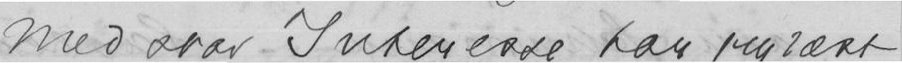Med stor Interesse har jeg læst
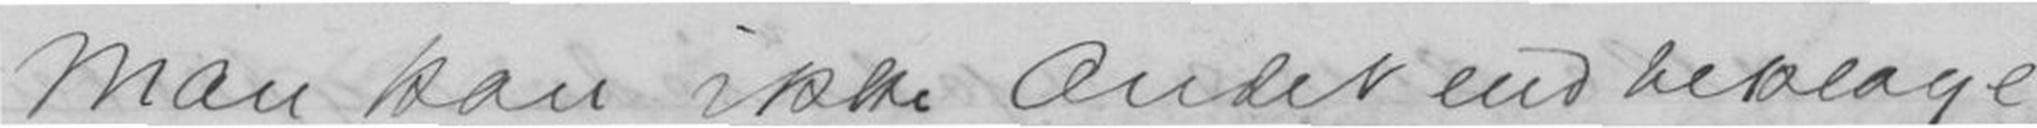Man kan ikke Andet end beklage,
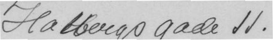Holbergsgade 11
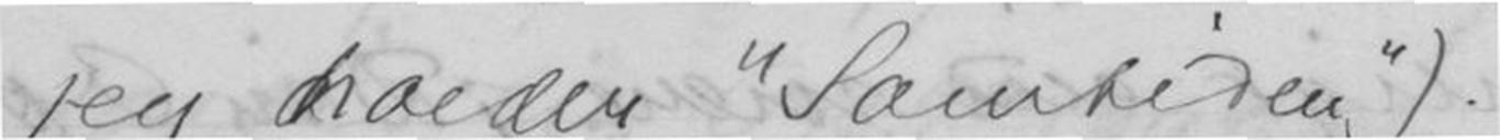jeg holder "Sannheden")
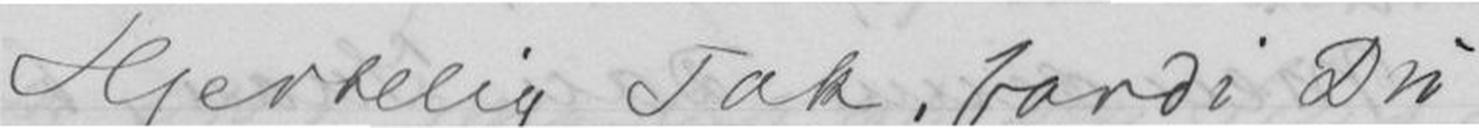Hjertelig tak, fordi Du
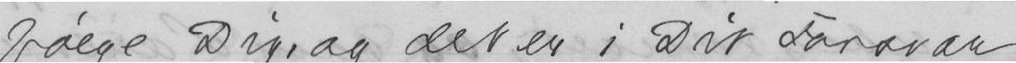følge Dig, og det er i Dit Forsvar
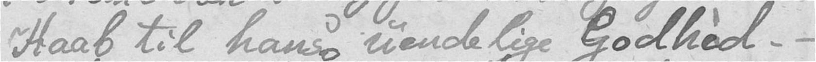Haab til hans uendelige Godhed. –
